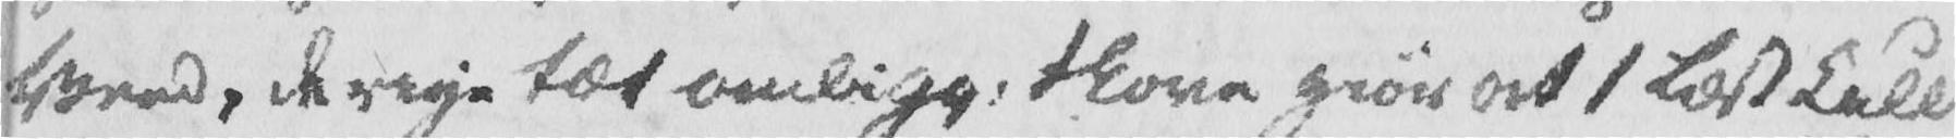Veed, de rige tæt omligg. Skove giør at 1 Læst Kull
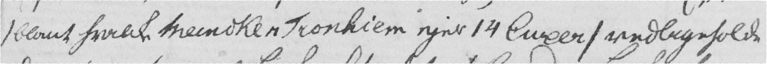/ blant hvilke Meincke i Tronhiem ejer 14 Cuxer / vedligeholde
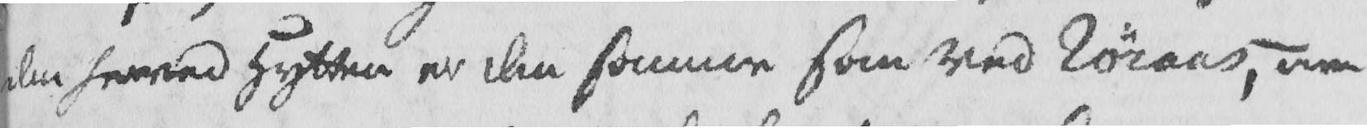den herved Hytten er den samme som ved Røraas, men
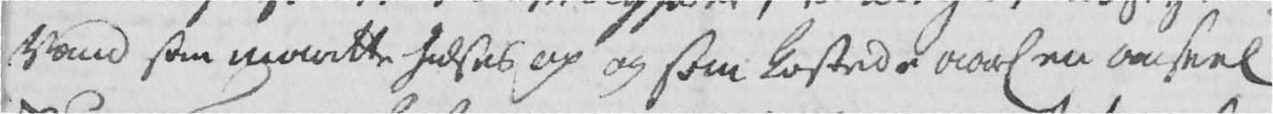Vand som maatte hidses op og som kostede aarl en anseel
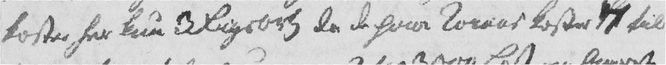koster her kun 3 Rigsort da de paa Røraas koster 7 til
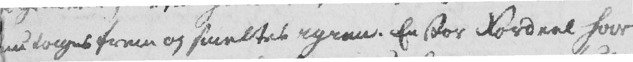nu tages frem og smeltes igien. En stor Fordeel har
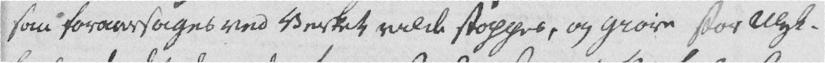som foraarsages ved Verket vilde stoppes, og giøre stor Ulyk-
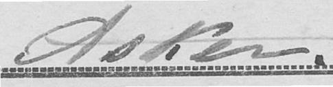Asker.
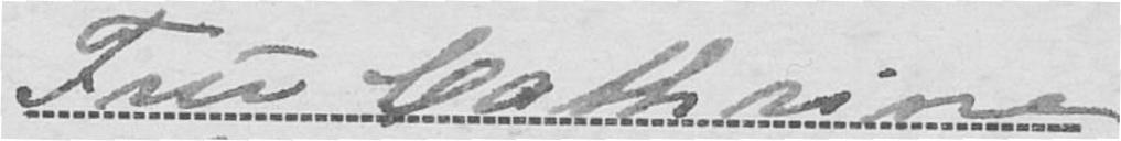Fru Cathrine
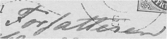Forfatteren
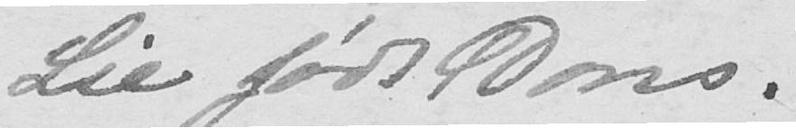Lie født Dons.
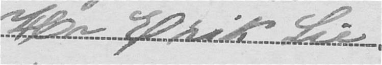Hr Erik Lie
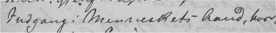Indgang i Menneskets Aand, hvor-
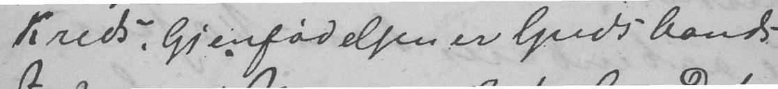Kreds Gjenførelsen er Guds Aands
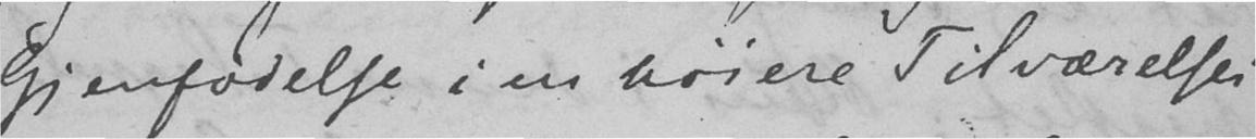Gjenfødelse i en høiere Tilværelses
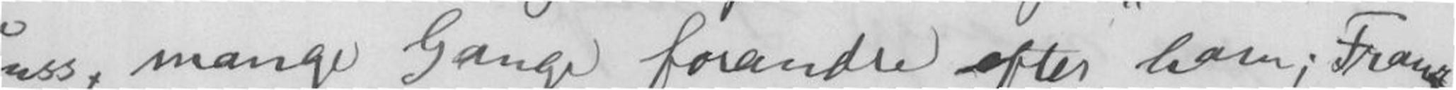mange Gange forandre efter ham; Franz
